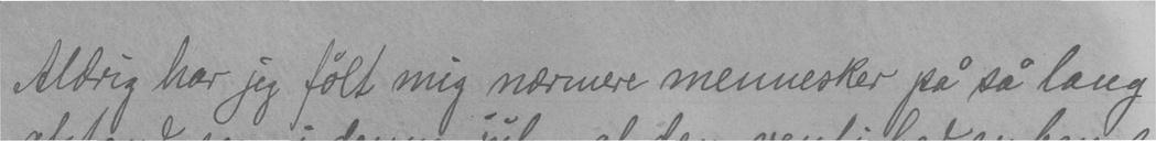Aldrig har jeg følt mig nærmere mennesker på så lang
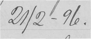21/2-96.
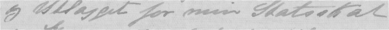og Utlægget for min Statsskat
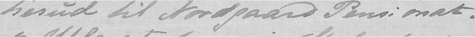herud til Nordgaard Pensionat.
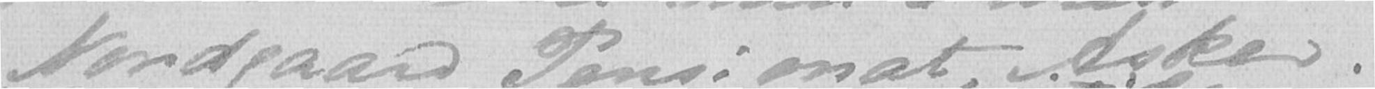Nordgaard Pensionat Asker.
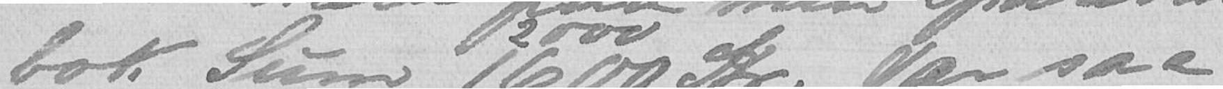bok Sum 1600 Kr. Vær saa
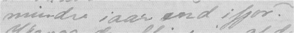mindre iaar end ifjor?
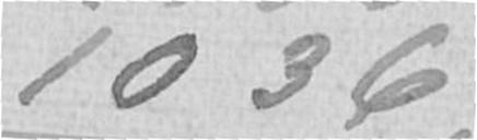1036
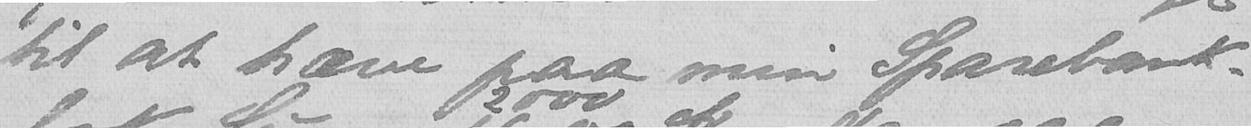til at hæve paa min Sparebank-
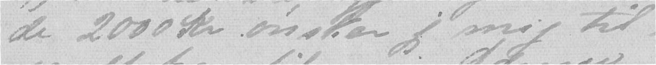de 2000 Kr ønsker jeg mig til-
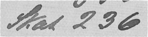Skat 236
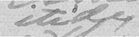itide
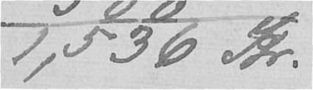1,536 Kr.
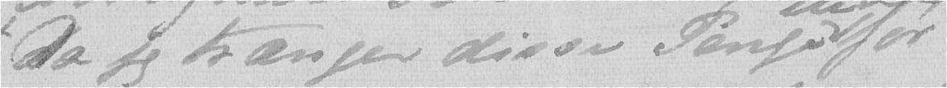da jeg trænger disse Penge for
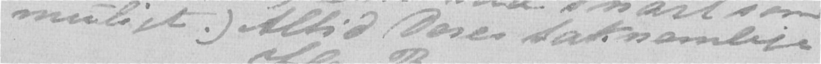muligt.) Altid Deres taknemlige
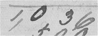1,036
